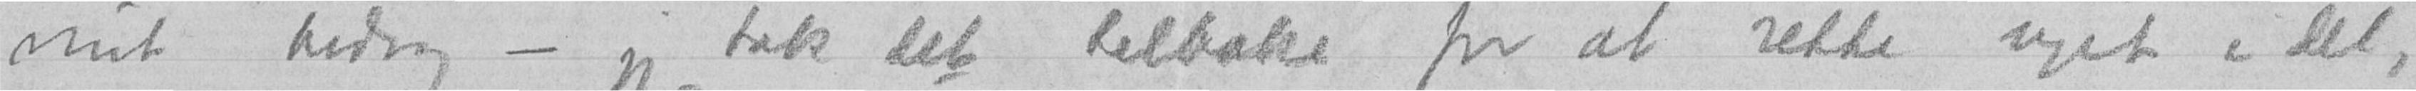mit bidrag - jeg tok det tilbake for at rette noget i det,
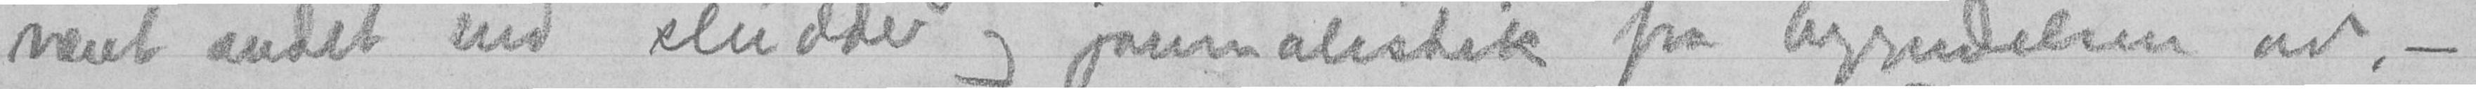været andet end sludder og journalistik fra begyndelsen av, -
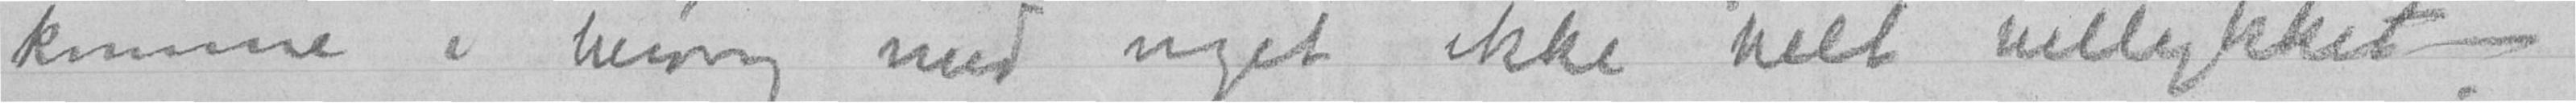komme i berøring med noget ikke helt vellykket -
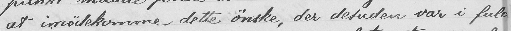at imødekomme dette ønske, der desuden var i fuld
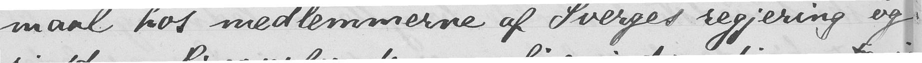maal hos medlemmerne af Sverges regjering og
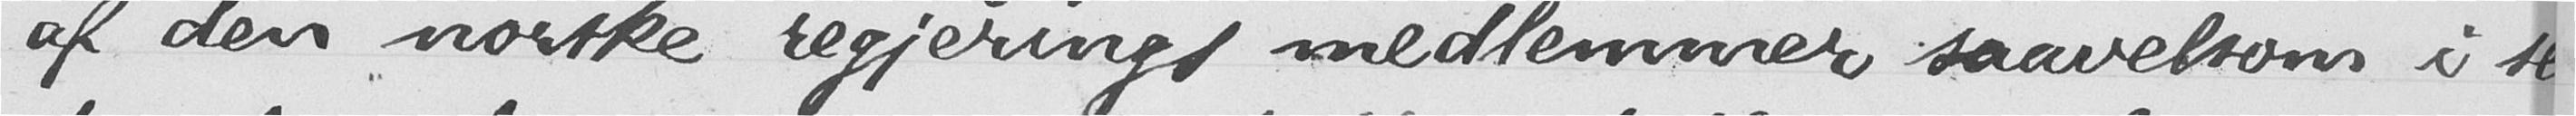af den norske regjerings medlemmer saavelsom i se
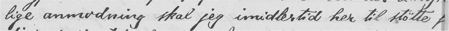lige anmodning skal jeg imidlertid her til støtte for
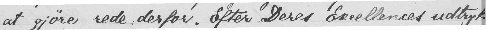at gjøre rede derfor. Efter Deres Excellences udtrykke-
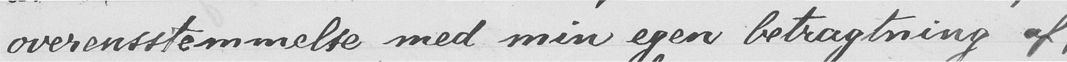overensstemmelse med min egen betragtning af,
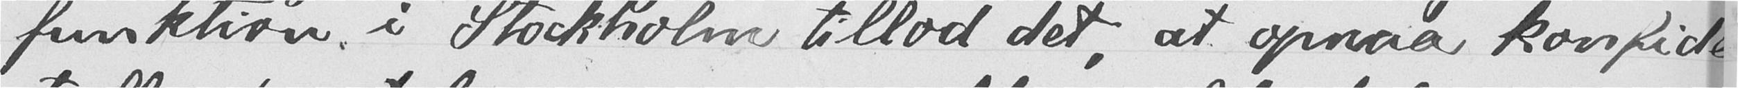funktion i Stockholm tillod det, at opnaa konfiden-
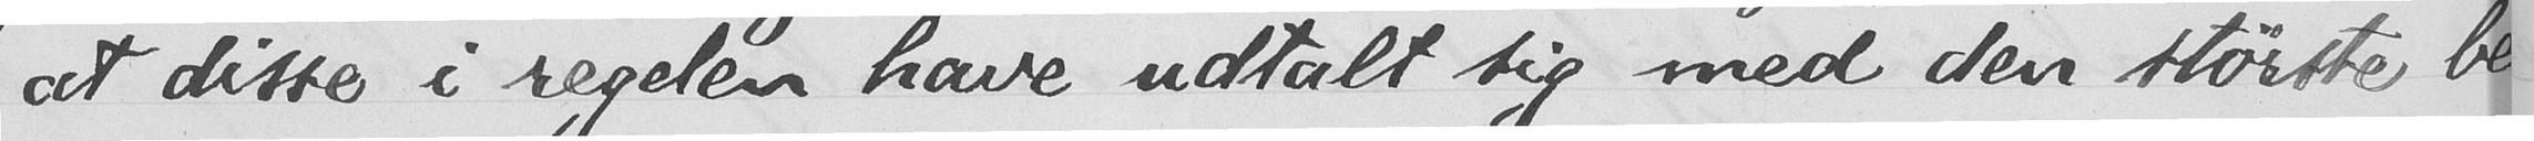at disse i regelen have udtalt sig med den største be
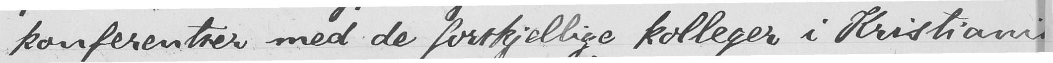konferentser med de forskjellige kolleger i Kristiania
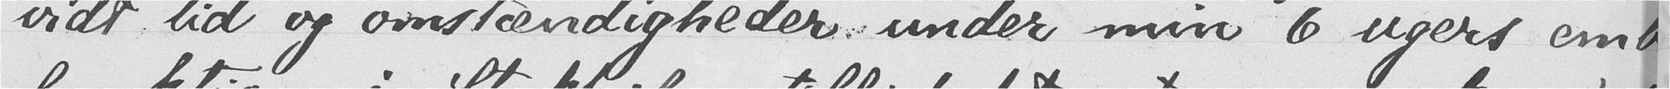vidt tid og omstændigheder under min 6 ugers emb
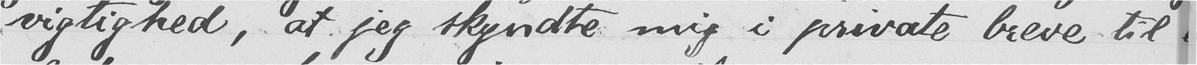vigtighed, at jeg skyndte mig i private breve til
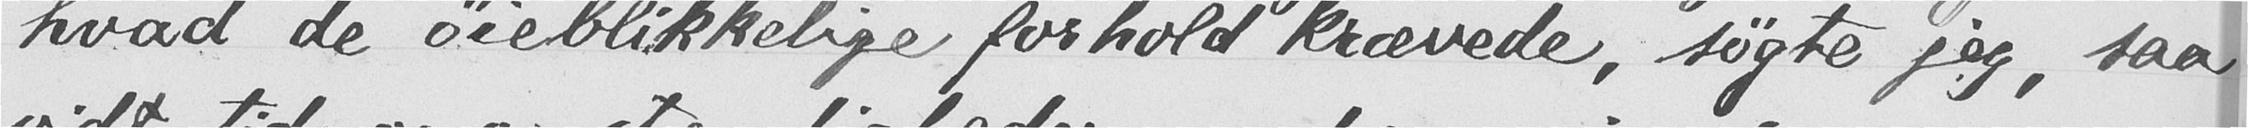hvad de øieblikkelige forhold krævede, søgte jeg, saa-
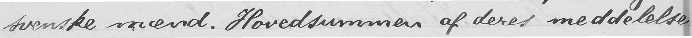svenske mænd. Hovedsummen af deres meddelelser
